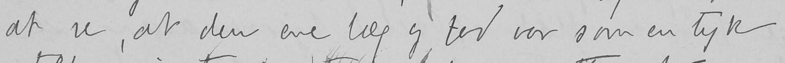at se, at den ene læg og fod var som en tyk
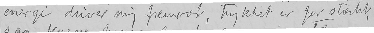energi driver mig fremover, trykket er for stærkt,
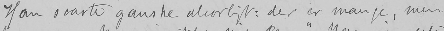Han svarte ganske alvorligt: der er mange, men
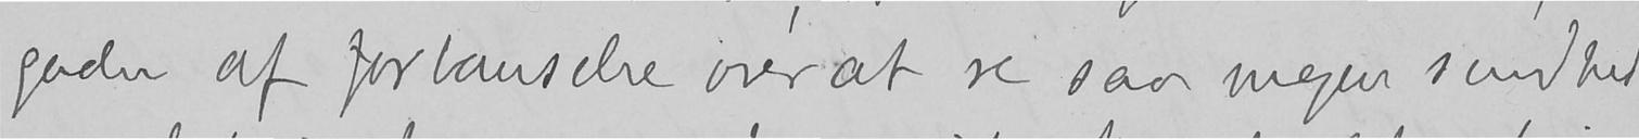gaden af forbauselse over at se saa megen sundhed
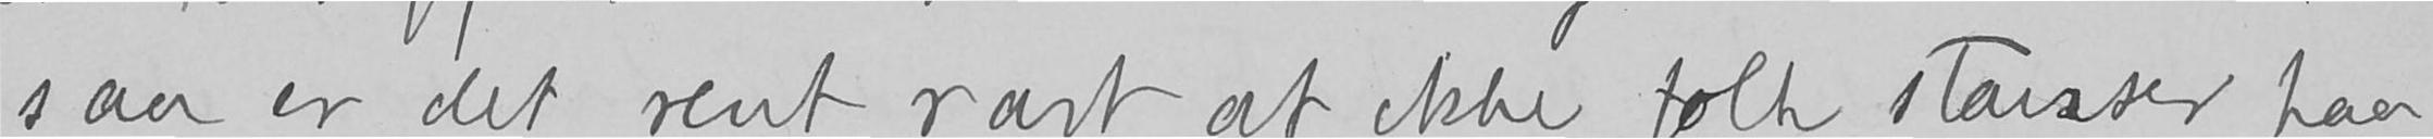saa er det rent rart at ikke folk stanser paa
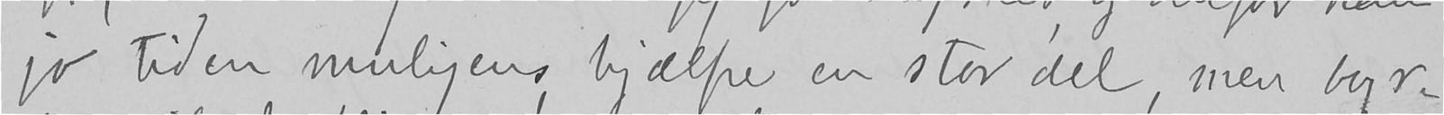jo tiden muligens hjælpe en stor del, men byr-
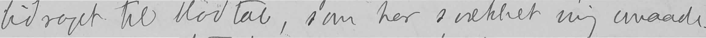bidraget til blodtab, som har svækket mig umaade-
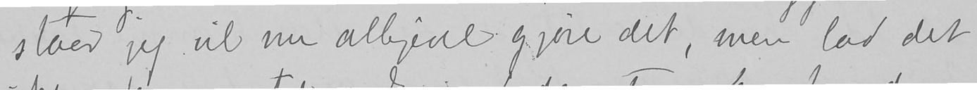staar jeg vil nu alligevel gjøre det, men lad det
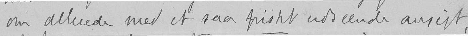om allerede med et saa friskt udseende ansigt,
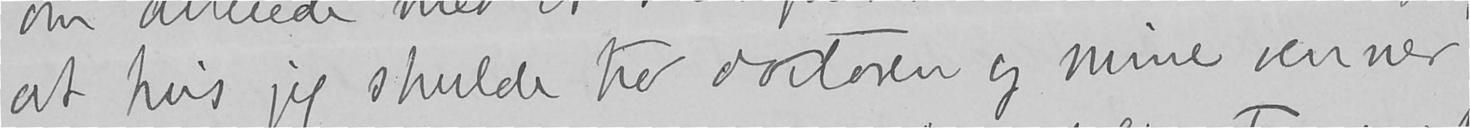at hvis jeg skulde tro doctoren og mine venner,
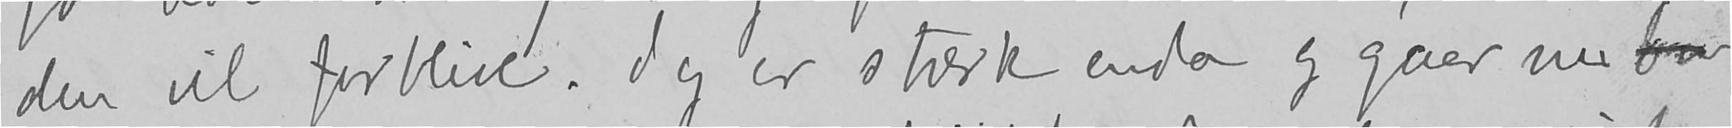den vil forblive. Jeg er stærk enda og gaar nu for
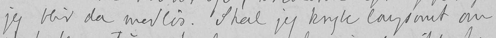jeg blir da modløs. Skal jeg krybe langsomt om
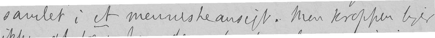samlet i et menneskeansigt. Men kroppen liger
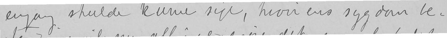engang skulde kunne sige, hvori ens sygdom be-
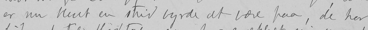er nu blevet en strid byrde at bære paa, de har
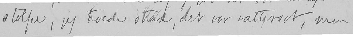stolpe, jeg troede strax, det var vattersot, men
